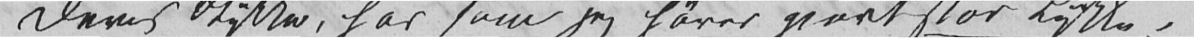Deres Stykke, har som jeg hører gjort stor Lykke,
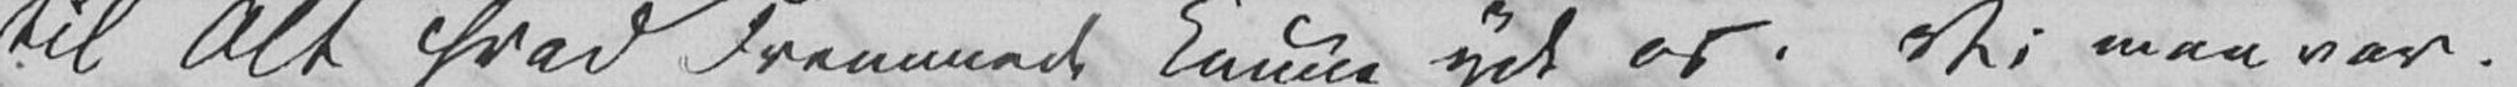til Alt hvad Fremmede kunne yde os. Vi maa var¬
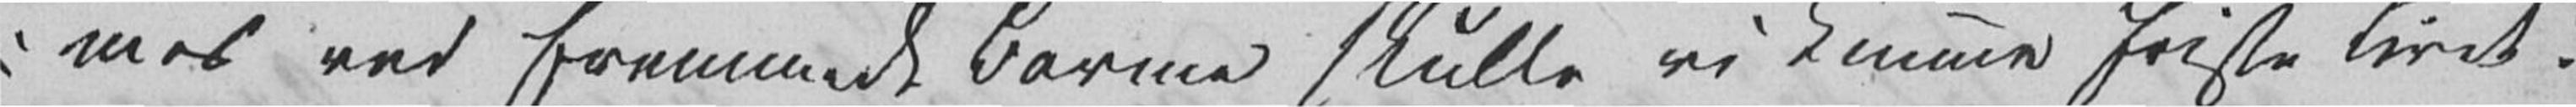mes ved fremmede Barme skulle vi kunne friste Livet.
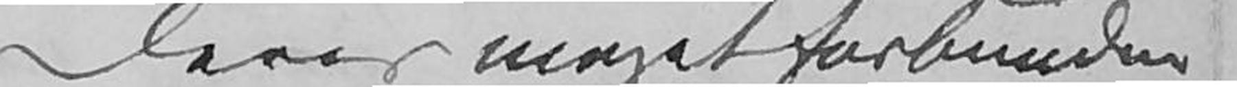Deres meget forbundne
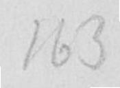163
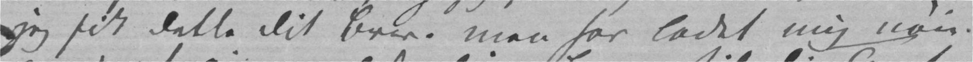jeg fik dette Dit Brev. men har ladet mig nøie
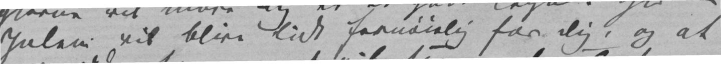Julen vil blive lidt fornøielig for Dig, og at
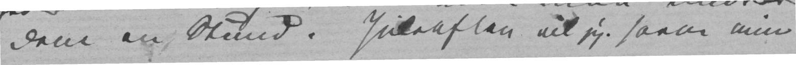dem en Stund. Juleaften vil jeg savne min
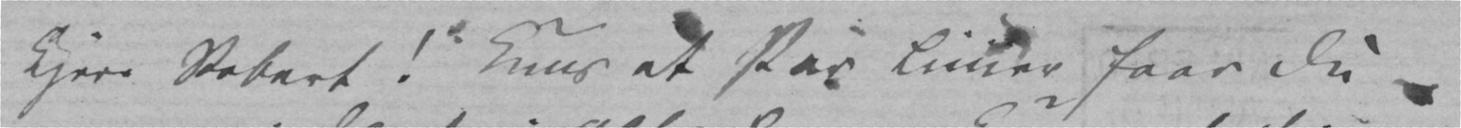Kjere Robert! Kuns et Par Linier faar Du
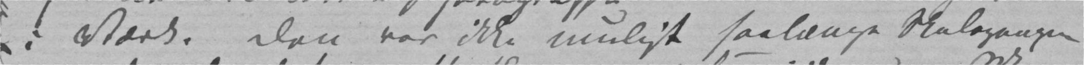i Værk. Den var ikke muligt saalænge Skolegangen
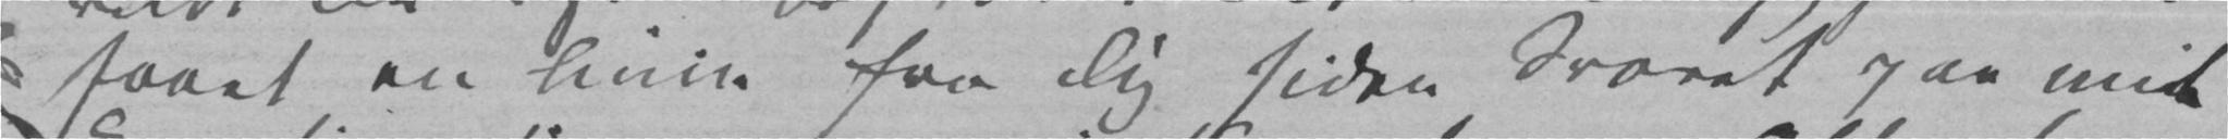faaet en Linie fra Dig siden Svaret paa mit
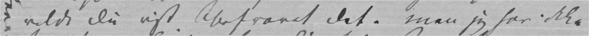vilde Du vist besvaret det. men jeg har ikke
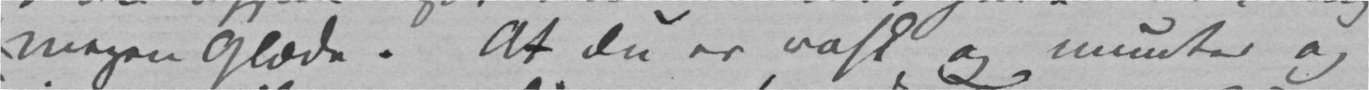megen Glæde. At Du er rask og munter og
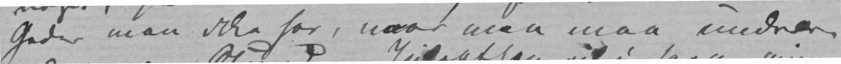Goder man ikke har, naar man maa undvære
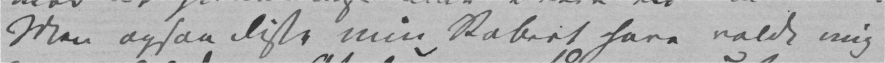Men ogsaa disse min Robert have voldt mig
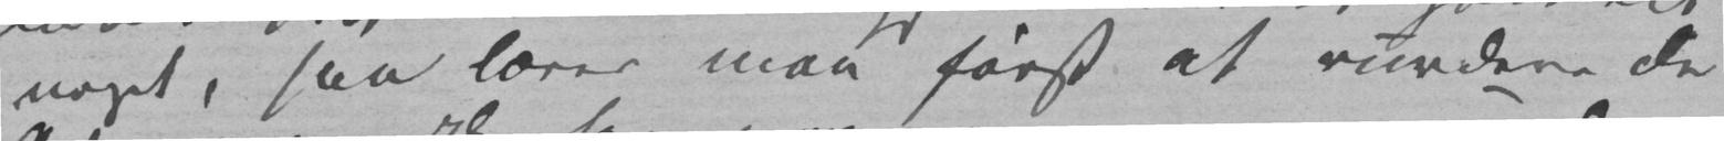noget, saa lærer man først at vurdere de
